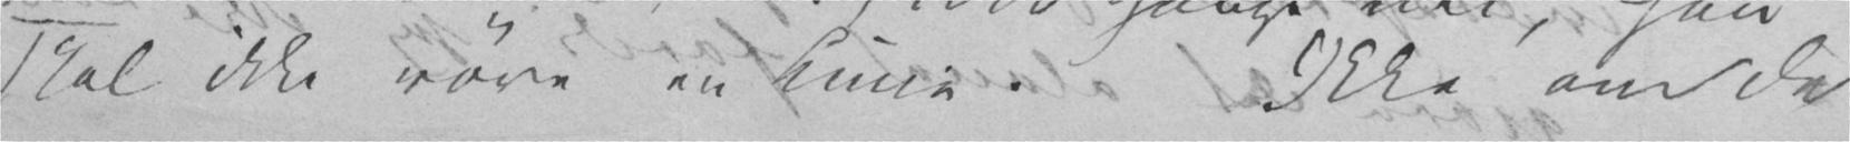skal ikke røre en Linie. Ikke om De
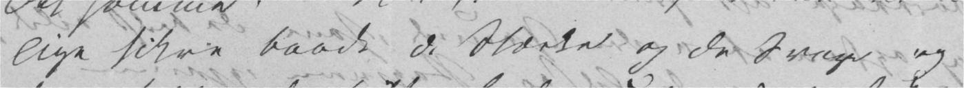lige sikre baade de Stærke og de Svage og
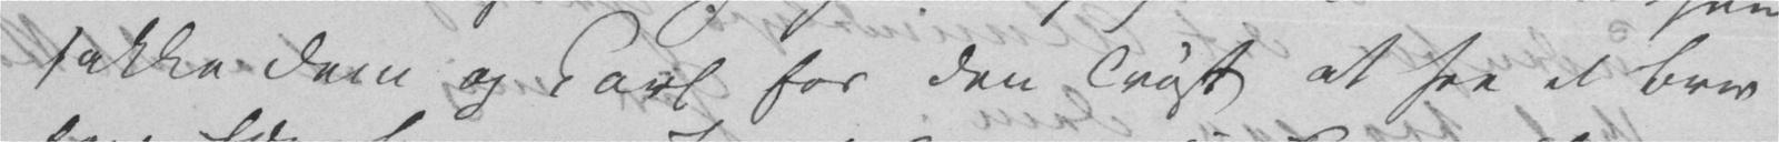takke Dem og Carl for den Trøst at see et Brev
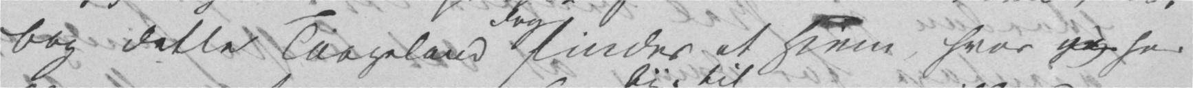bag dette Taageland dog findes et Hjem, hvor man har
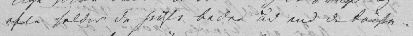ofte holder de sidste bedre ud end de første.
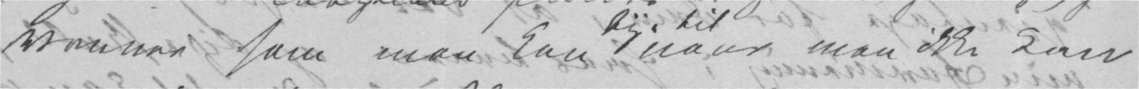Venner som man kan tye til naar man ikke kan
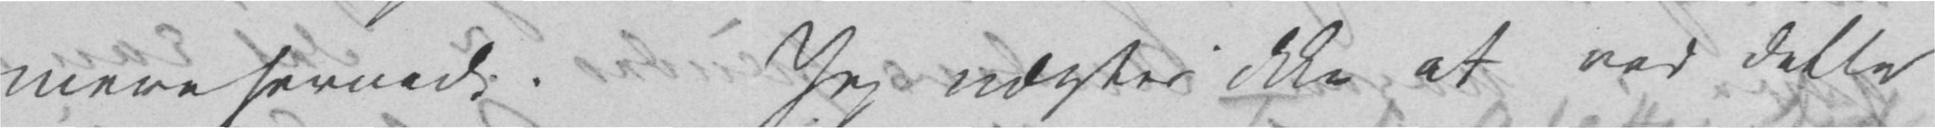mere hernede. Jeg nægter ikke at ved dette
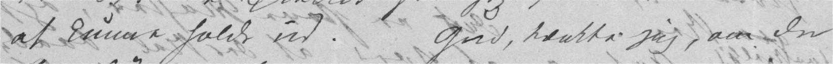at kunne holde ud. Gud, tænkte jeg, om de
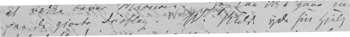paa de gjorte Forslag. W. skulde yde sin Hjelp
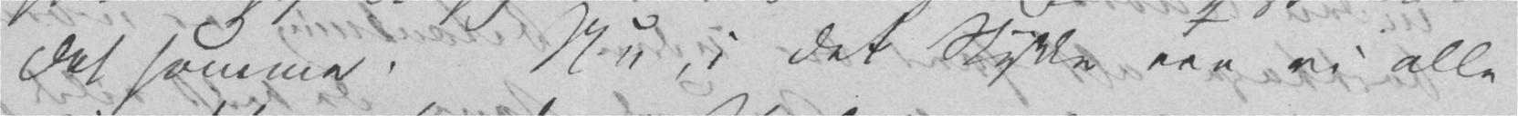det samme. Nu, i det Stykke ere vi alle
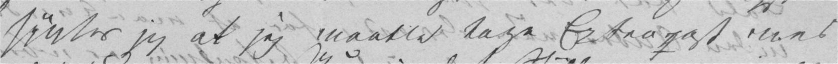syntes jeg at jeg maatte tage Extrapost med
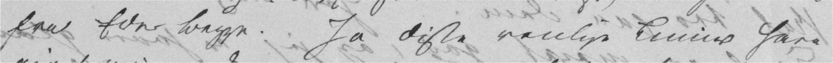fra Eder begge. Ja disse venlige Linier have
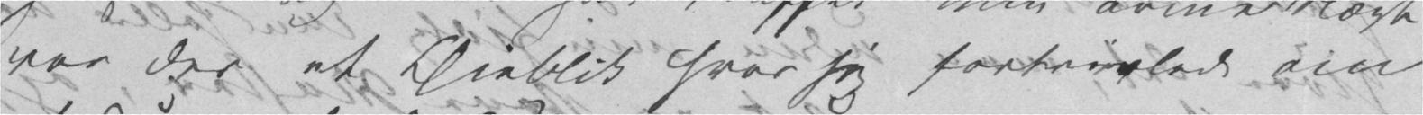var der et Øieblik hvor jeg fortvivlede om
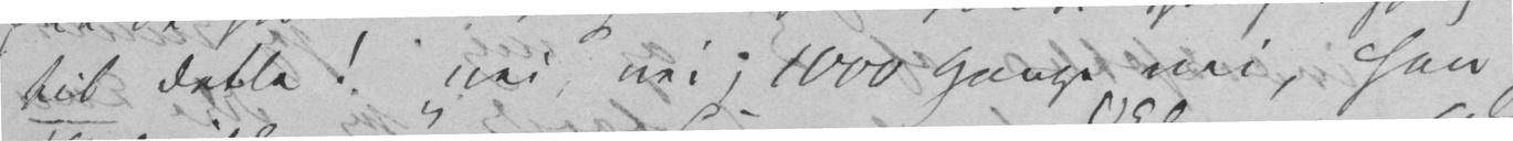til dette! nei, nei; 1000 Gange nei, han
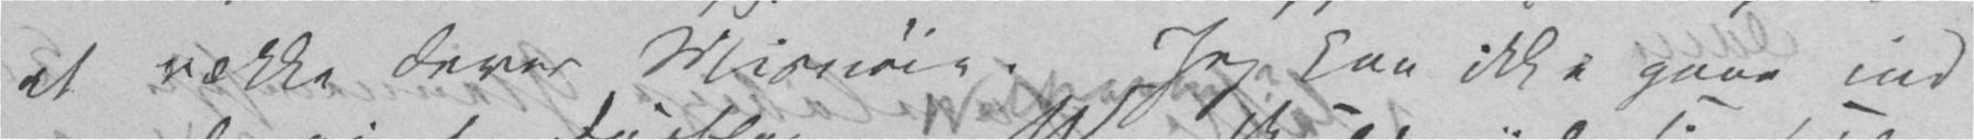at vække Deres Misnøie. Jeg kan ikke gaae ind
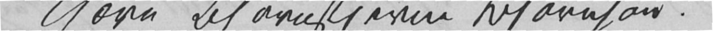Kjære Bjørnstjerne Bjørnson!
